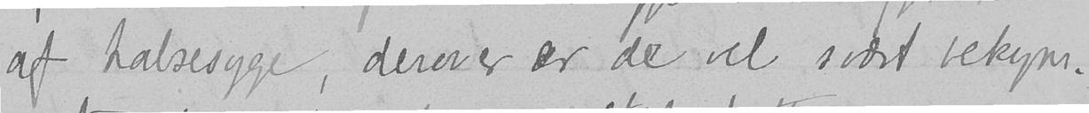af halsesyge, derover er de vel svært bekym-
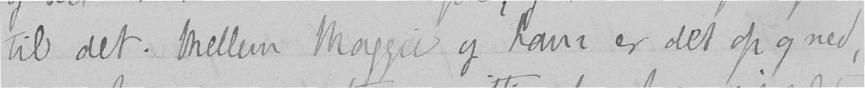til det. Mellem Maggie og ham er det op og ned,
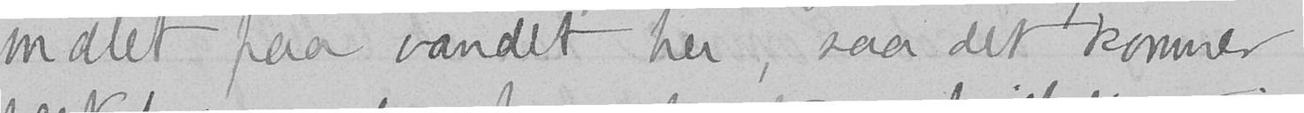malet paa vandet her, saa det kommer
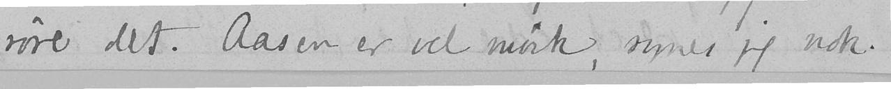røre det. Aasen er vel mørk, synes jeg nok.
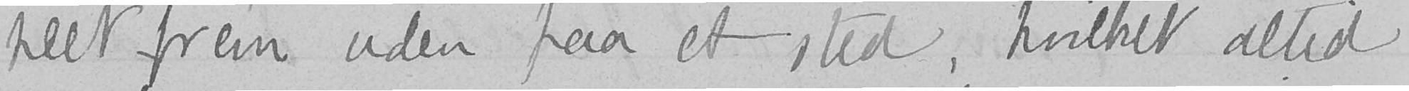helt frem uden paa et sted, hvilket altid
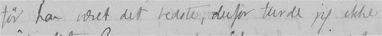før har været det bedste, derfor turde jeg ikke
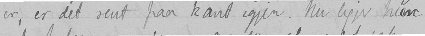er, er det rent paa kant igjen. Nu ligger hun
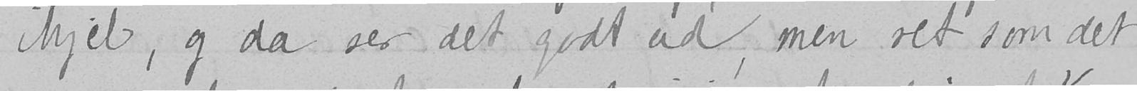ihjel, og da ser det godt ud, men ret som det
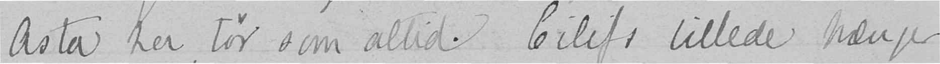Asta her, tør som altid. Eilifs billede hænger
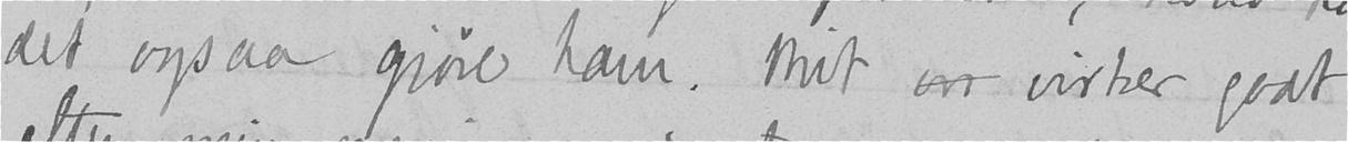det ogsaa gjøre ham. Mit on virker godt
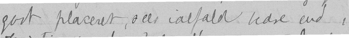godt placeret, sees ialfald bedre end i
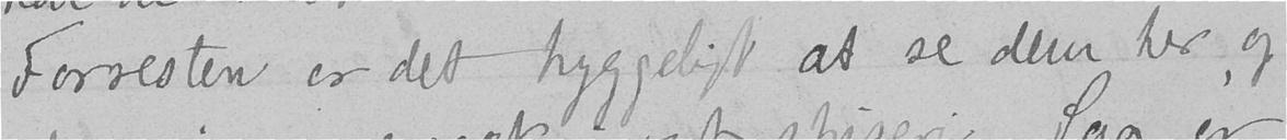Forresten er det hyggeligt at se dem her, og
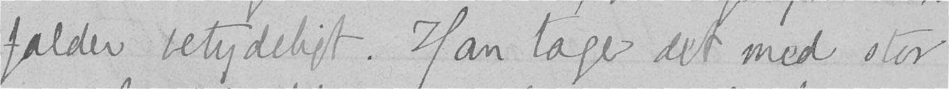falder betydeligt. Han tager det med stor
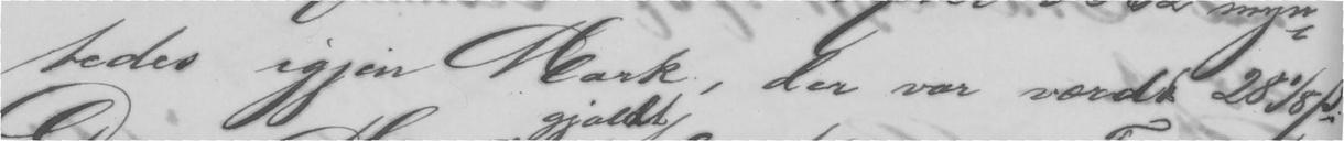tedes igjen Mark, der var værdt 28⅛ ẞ.
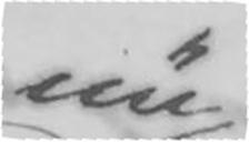nu
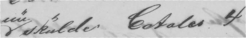skulde betales 4
